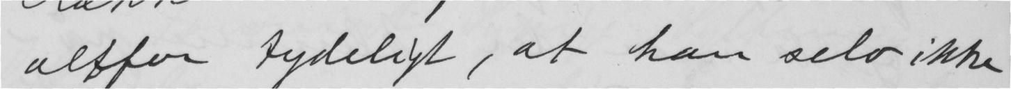altfor tydeligt, at han selv ikke
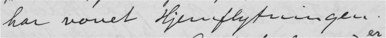har vovet Hjemflytningen.
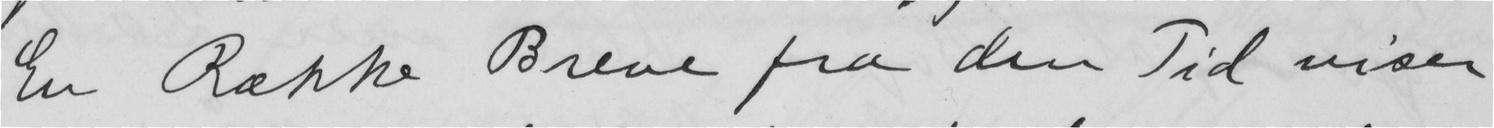En Række Breve fra den Tid viser
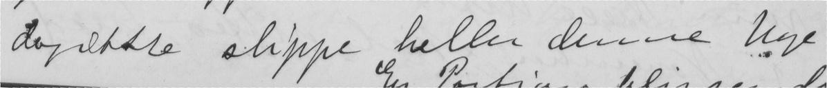dogstste slippe heller denne Uge
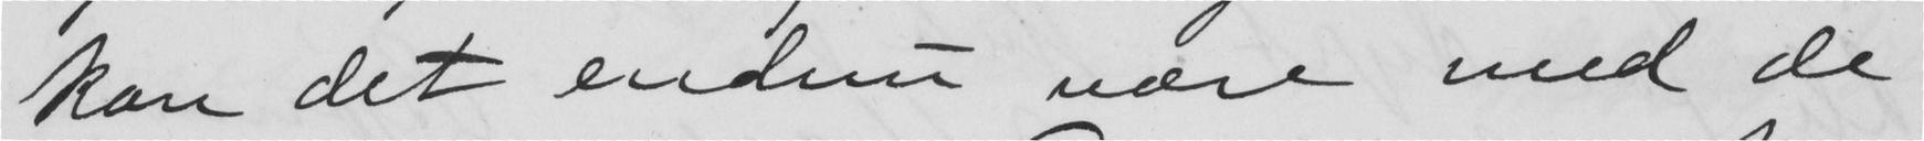kan det endnu være med de
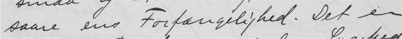saare ens Forfængelighed. Det er
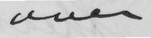over
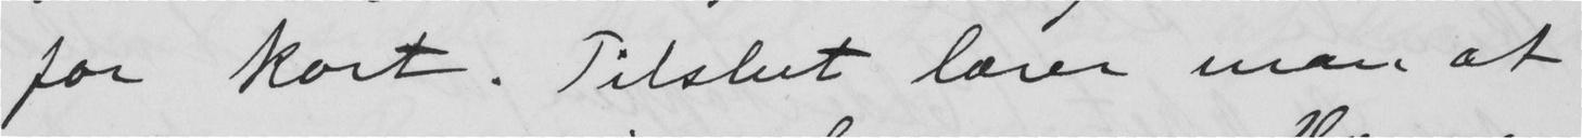for kort. Tilslut lærer man at
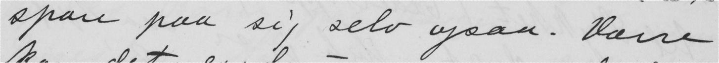spare paa sig selv ogsaa. Værre
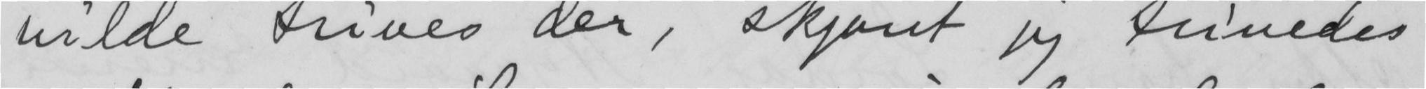vilde trives der, skjønt jeg trivedes
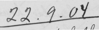22.9.04
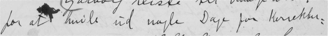for att hvile ud nogle Dage for Korektu-
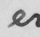er
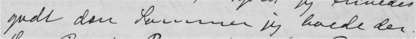godt den sommer jeg boede der.
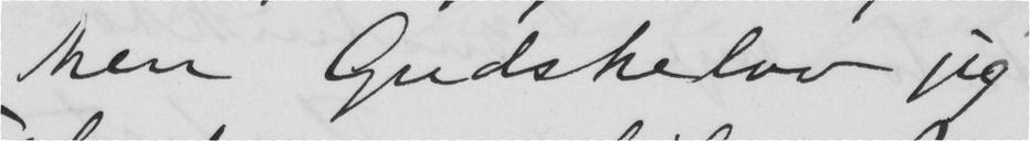Men Gudskelov jeg
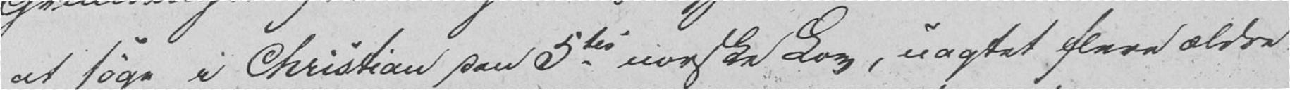at søge i Christian den 5tes norske Lov, uagtet flere ældre
